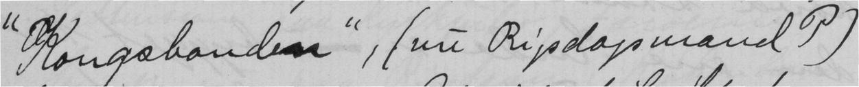"Kongsbanden", (nu Rigsdagsmand P)
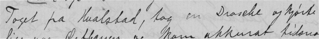Toget fra Hvalstad, tog en Drosche og kjørte
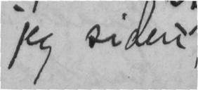jeg siden
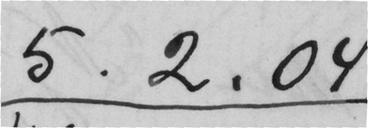5.2.04
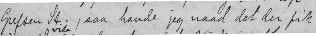Grefsen St., saa havde jeg naad det der fik
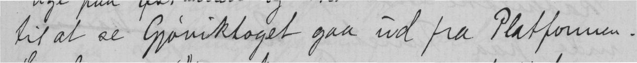til at se Gjøviktoget gaa ud fra Platformen.
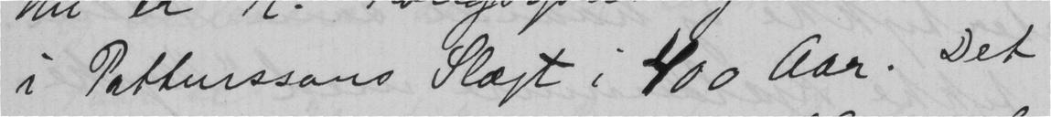i Patturssons Slægt i 400 aar. Det
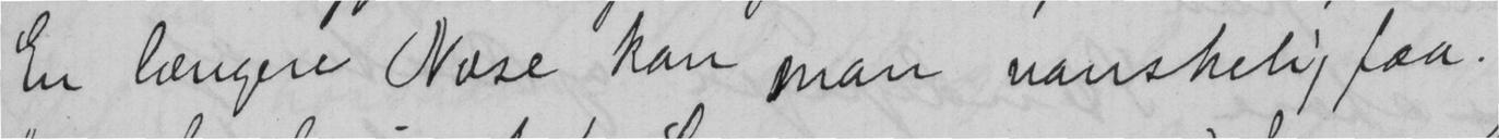En længere Næse kan man vanskelig faa.
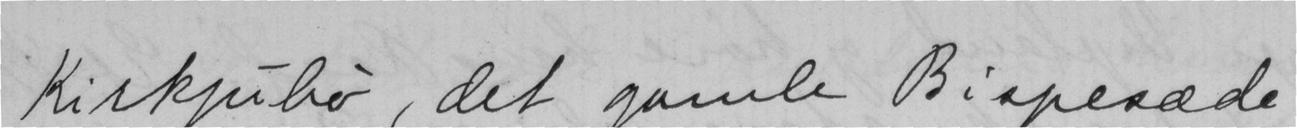Kirkjubø, det gamle Bispesæde
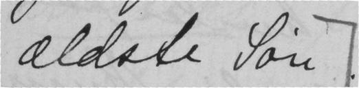ældste Søn.
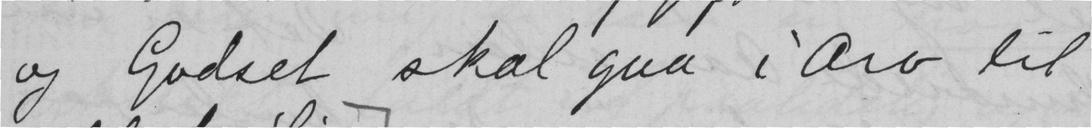og Godset skal gaa i Arv til
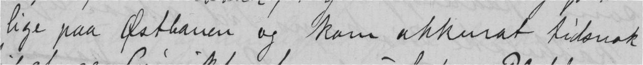lige paa Østbanen og kom akkurrat tidsnok
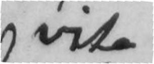vite
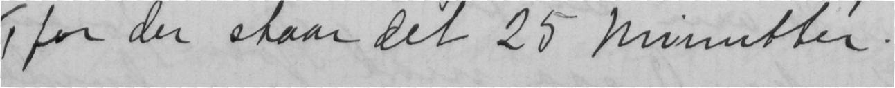, for der staar det 25 Minutter.
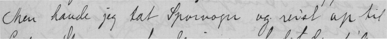Men havde jeg tat Sporvogn og reist op til
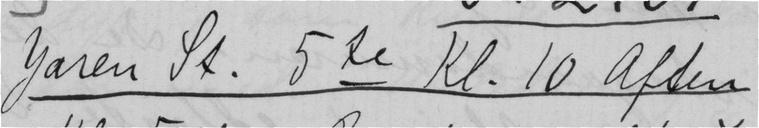Jaren St. 5te Kl. 10 Aften
